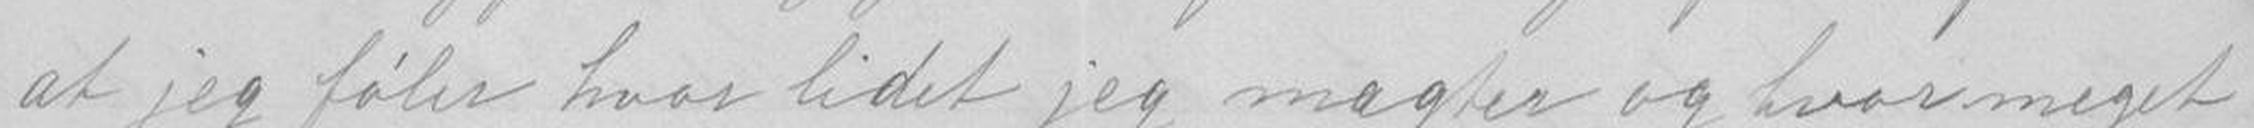at jeg føler vor lidet jeg magter og hvor meget
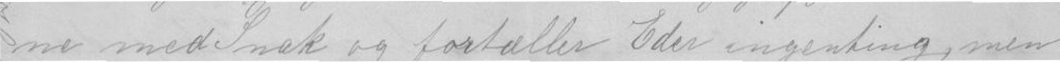ne med Smak og fortæller Eder ingenting, men
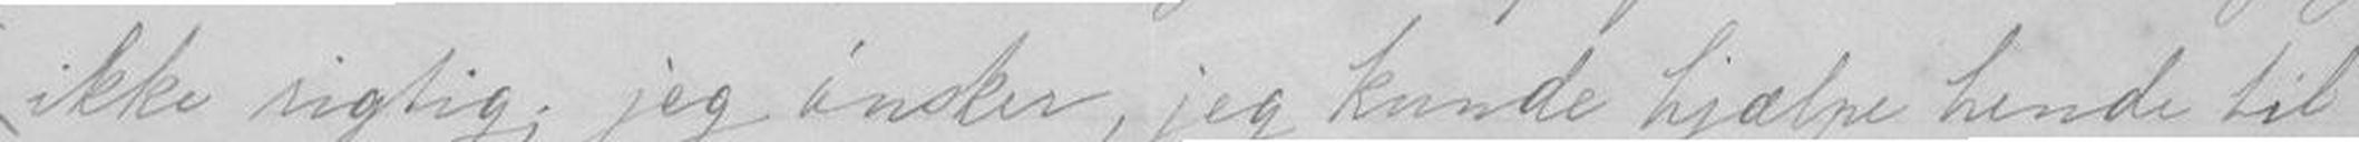ikke rigtig; jeg ønsker, jeg kunde hjælpe hende til
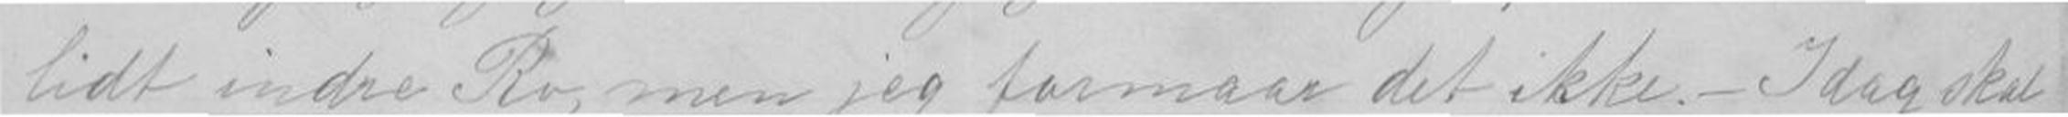lidt indre Ro, men jeg formaar det ikke.- Idag skal
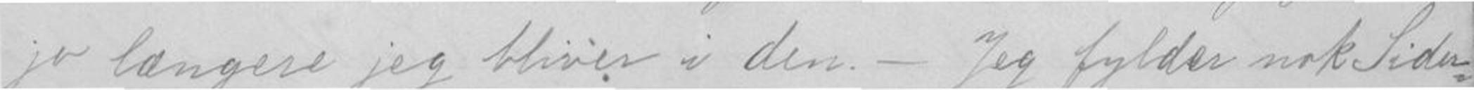jo længere jeg bliver i den.- Jeg fylder nok Sider-
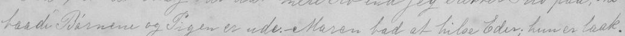baade Børnene og Pigen er ude.- Maren bad at hilse Eder; hun er laak.
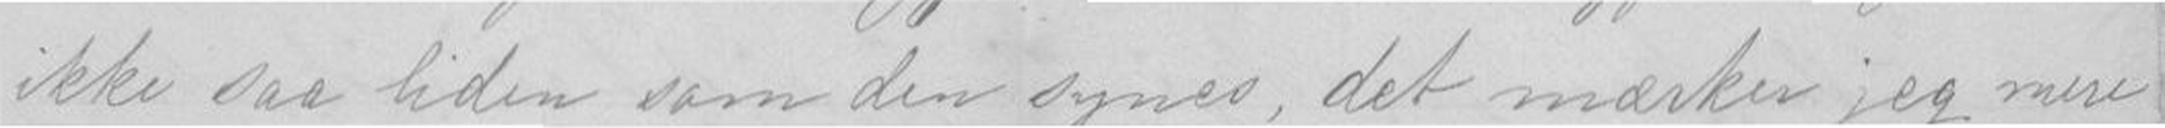ikke saa liden som den synes, det mærker jeg mere
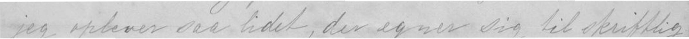jeg oplever saa lidet, der egner sig til skriftlig
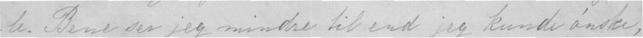le. Bene ser jeg mindre til end jeg kunde ønske,
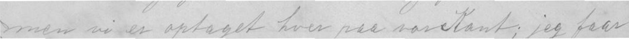men vi er optaget hver paa vor kant; jeg faar
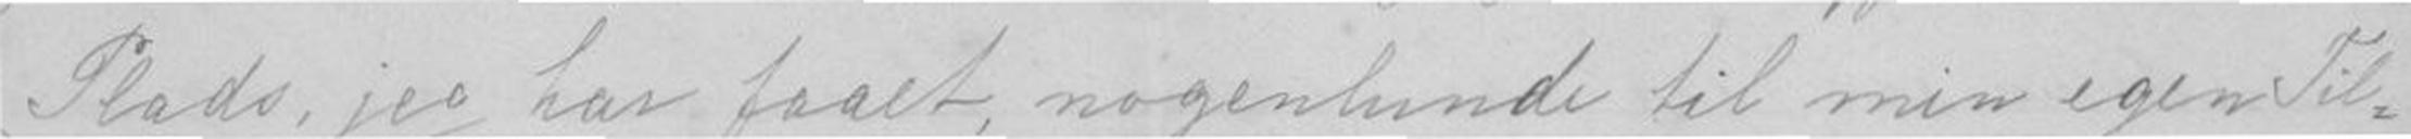Plads, jeg har faaet, nogenlunde til min egen Til-
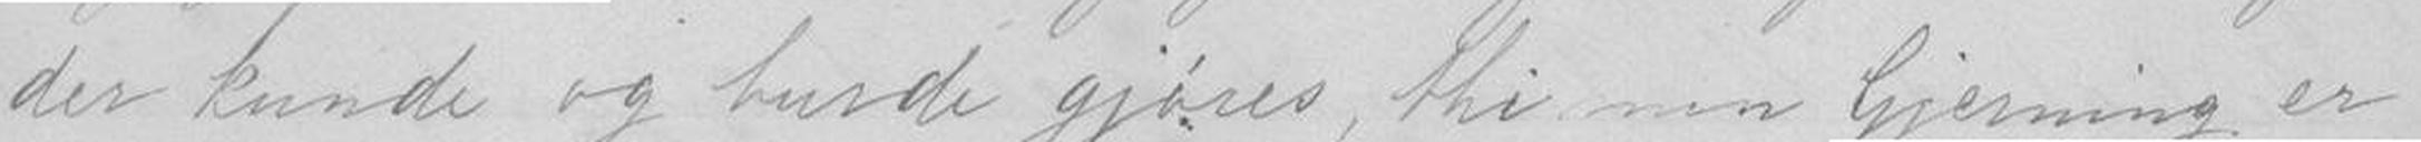der kunde og burde gjøres, thi min Gjerning er
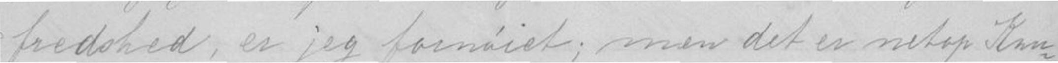fredshed, er jeg fornøiet; men det er netop Kun-
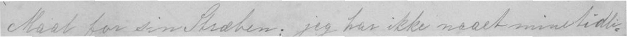Maal for sin Stræben, jeg har ikke maaet mine tidli-
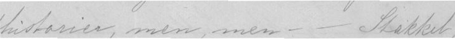historier, men, men Stakkel,
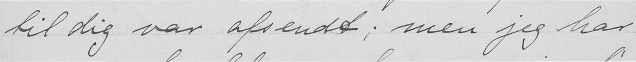til dig var afsendt; men jeg har
